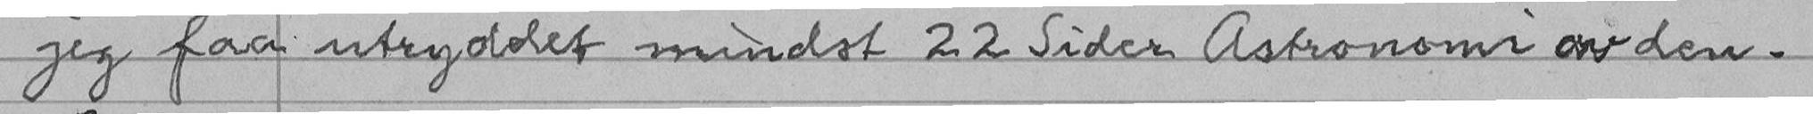jeg faa utryddet mindst 22 Sider Astronomi av den.
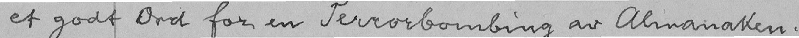et godt Ord for en Terrorbombing av Almanaken.
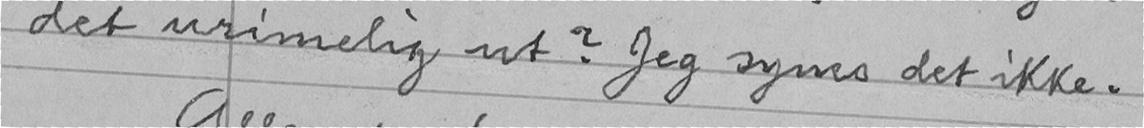det urimelig ut? Jeg synes det ikke.
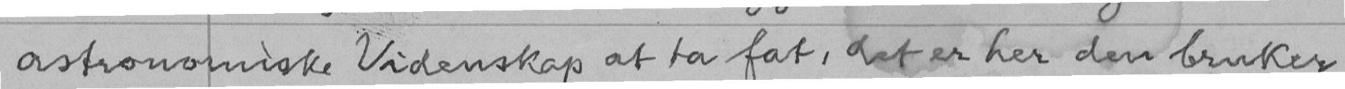astronomiske Videnskap at ta fat, det er her den bruker
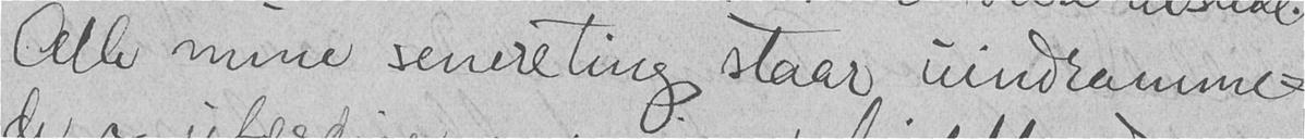Alle mine senere ting staar uindramme-
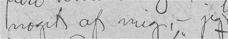noget af mig, - jeg
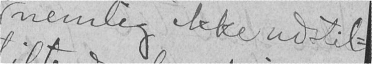nemlig ikke udstil-
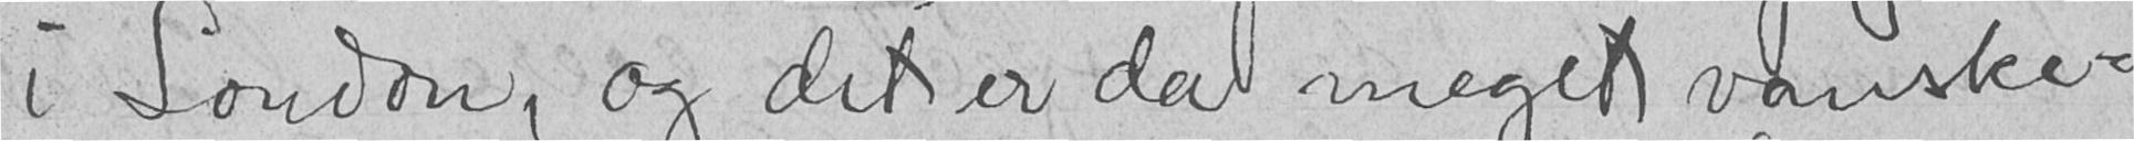i London, og det er da meget vanske-
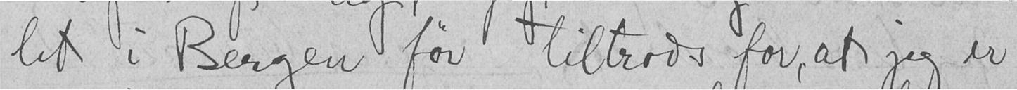let i Bergen før tiltrods for, at jeg er
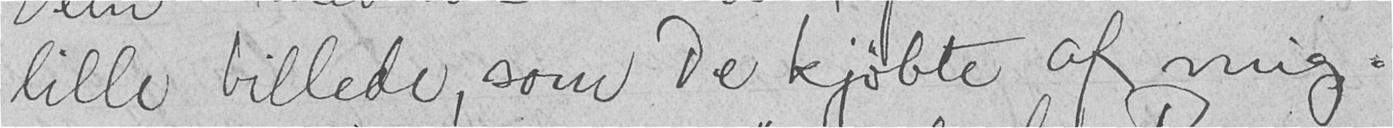lille billede, som De kjøbte af mig.
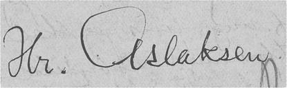Hr. Aslaksen.
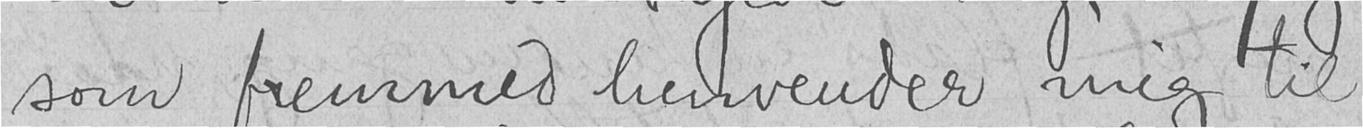som fremmed henvender mig til
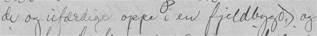de og ufærdige oppe i en fjeldbygd, og
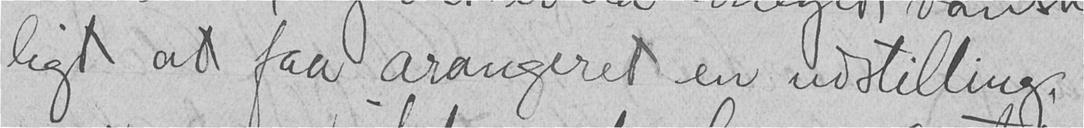ligt at faa arangeret en udstilling,
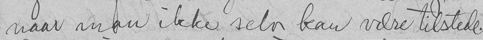naar man ikke selv kan være tilstede.
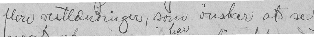flere vestlændinger, som ønsker at se
